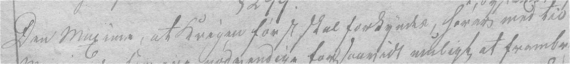Den Maxime, at Krigen først skal forkyndes, hører med til
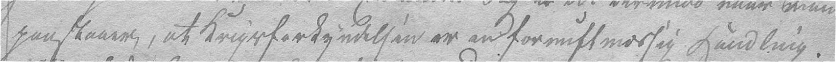paastaaer, at Krigsforkyndelsen er en fornuftmæssig Handling.
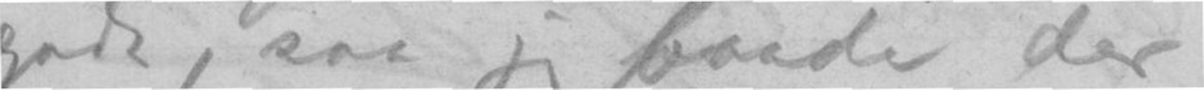godt, saa jeg baade der og
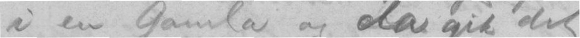i en Gamla og da gik det
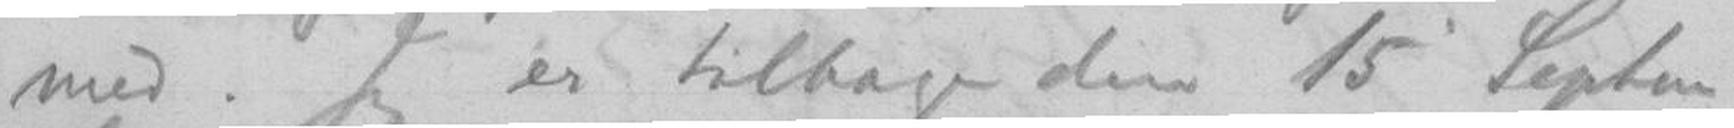med. Jeg er tilbage den 15 Septem-
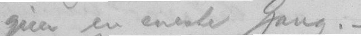gien en eneste Gang. -
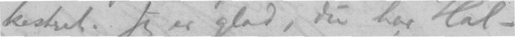kestret. Jeg er glad, du har Hal-
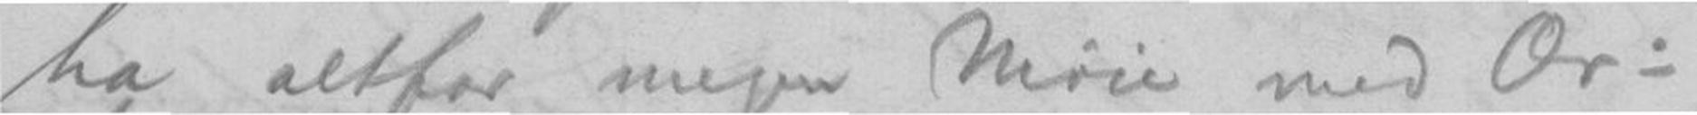ha altfor megen Møie med Or-
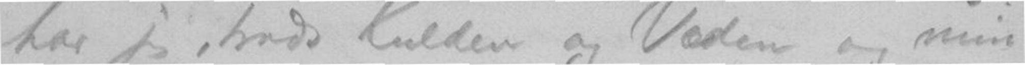har jeg, trods Kulden og Væden og mind-
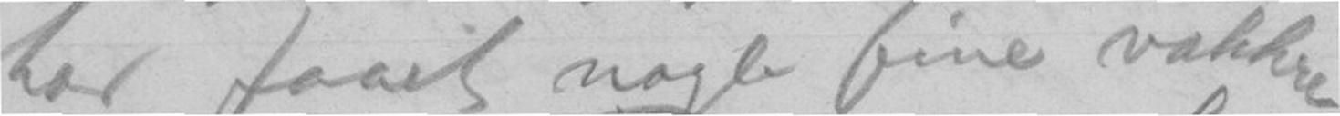har faaet nogle fine vakkre.
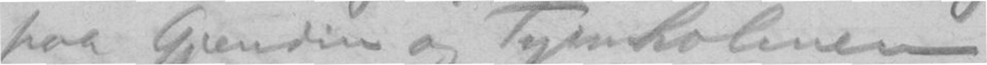paa gjendin og Tyinholmen
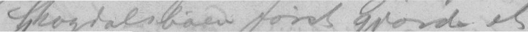Skogdalsbøen først gjorde et
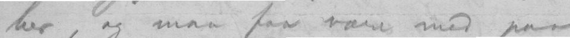ber, og maa faa være med paa
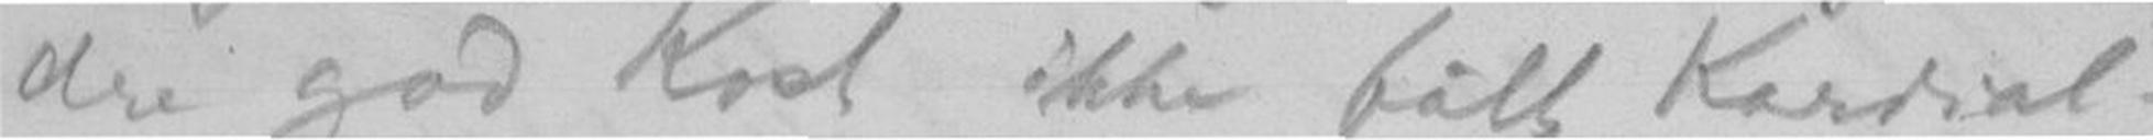re god Kost ikke følt Kardial-
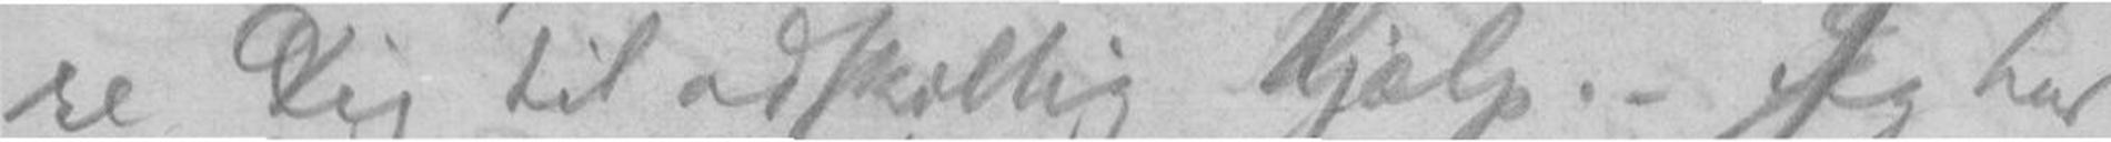re Dig til adskillig Hjælp. - Jeg har
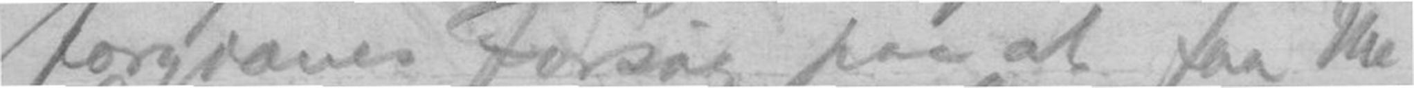forgjæves Forsøg paa at faa Me-
